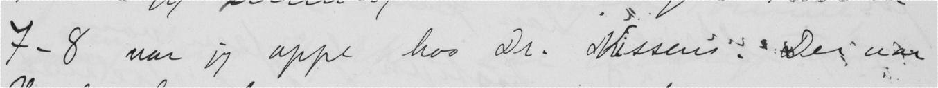7-8 var jeg oppe hos Dr. Nissens. Der var
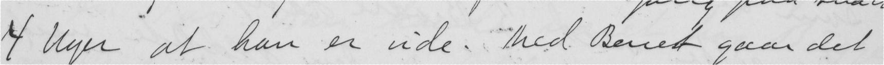4 Uger at han er ude. Med Benet gaar det
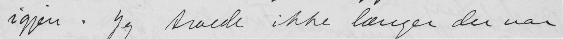igjen. Jeg troede ikke længer der var
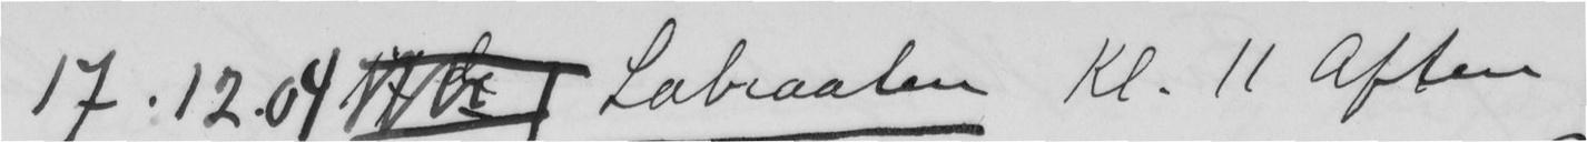17.12.04  Labraaten Kl. 11 Aften Mrdag
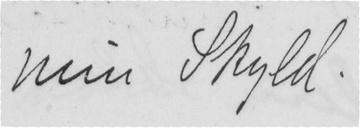Min Skyld.
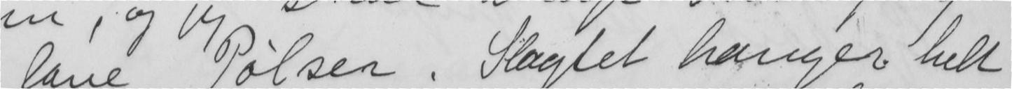lave Polser. Slagtet hænger helt
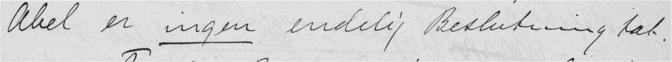Abel er ingen endelig Beslutning tat.
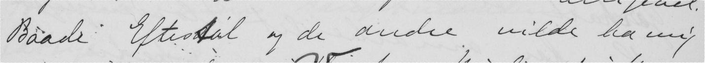Baade Eftestøl og de andre vilde ha mig
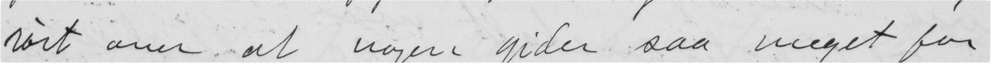rørt over at nogen gider saa meget for
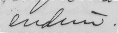endnu
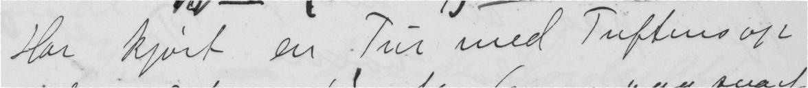Har kjørt en Tur med Tuftens op
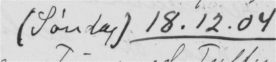(Søndag) 18.12.04
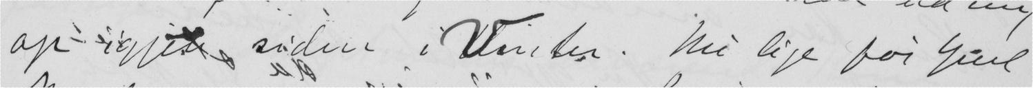op igjen siden i Vinter. Nu lige før Jul
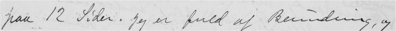paa 12 Sider. Jeg er fuld af Beundring, og
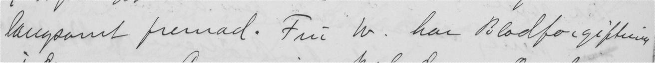langsomt fremad. Fru W. har Blodforgiftning
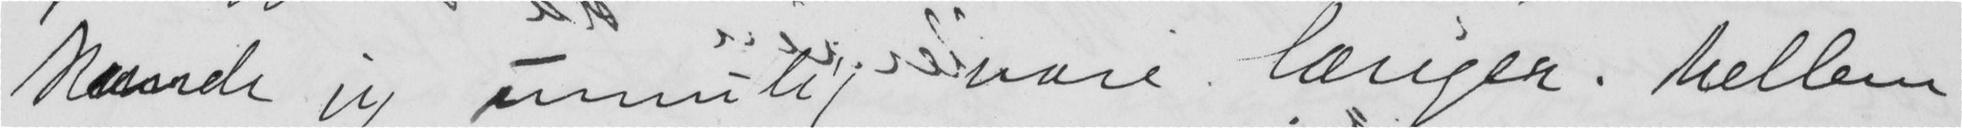kunde jeg umulig være længer. Mellem
